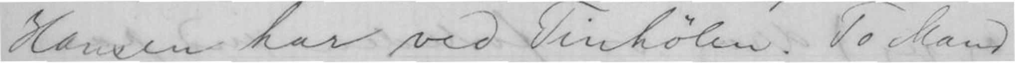Hansen har ved Tinhølen. To Mand
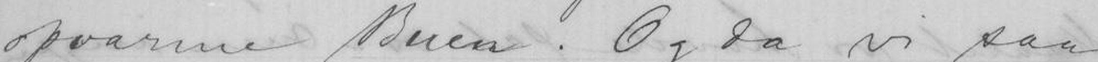opvarme Buen. Og da vi saa
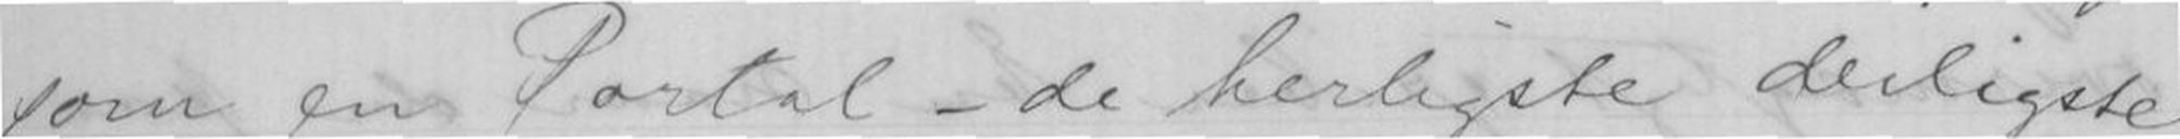som en Portal - de herligste deiligste
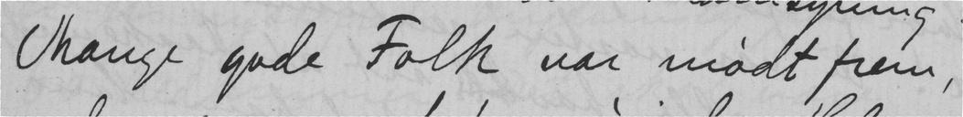Mange gode Folk var mødt frem,
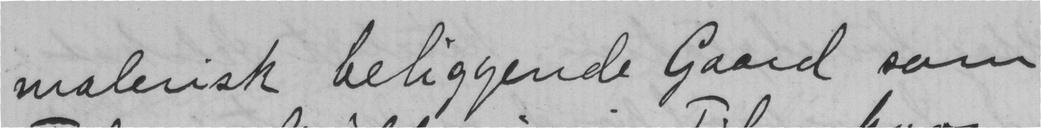malerisk beliggende Gaard som
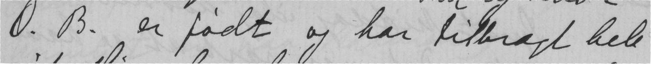O. B. er født og har tilbragt hele
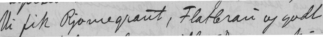Vi fik Rjomegraut, Flatbrau og godt
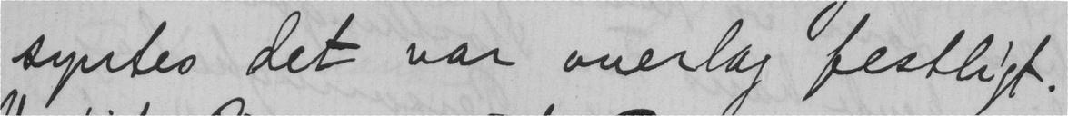syntes det var overlag festligt.
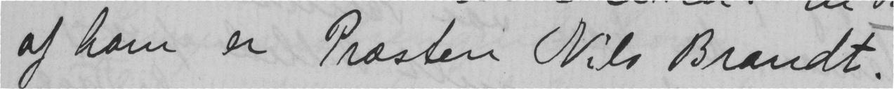af ham er Præsten Nils Brandt.
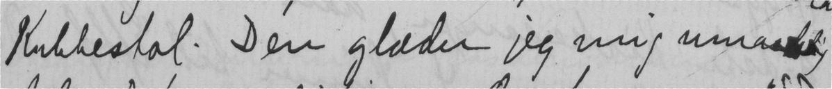Kubbestol. Den glæder jeg mig umaadelig
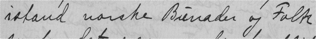i stand norske Bunader og Folk
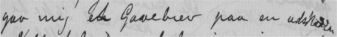gav mig et Gavebrev paa en udskaaren
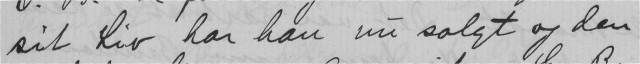sit Liv har han nu solgt og den
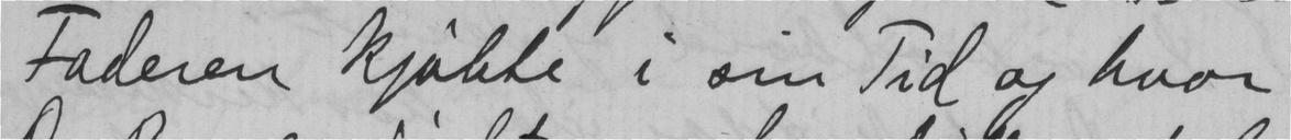Faderen kjøbte i sin Tid og hvor
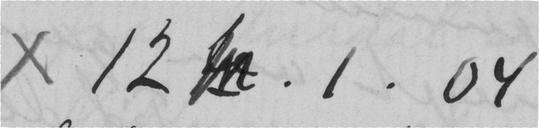x 12.1.04
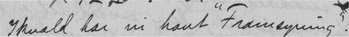I kvæld har vi havt "Framsyning".
